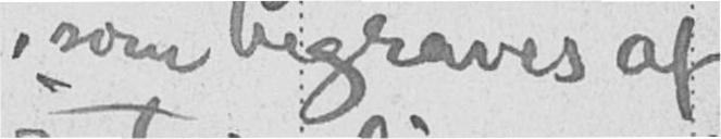, som begraves af
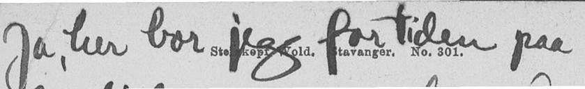Ja, her bor jeg for tiden paa
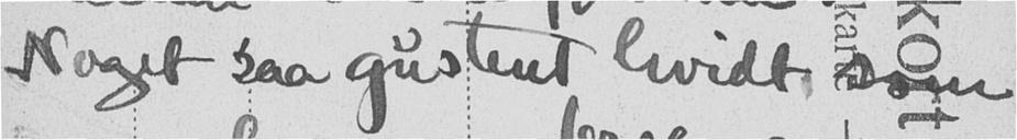Noget saa gustent hvidt som
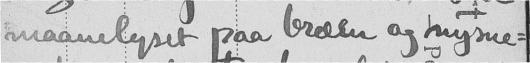maanelyset paa bræen og nysne-
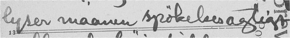lyser maanen spøkelsesagtigt
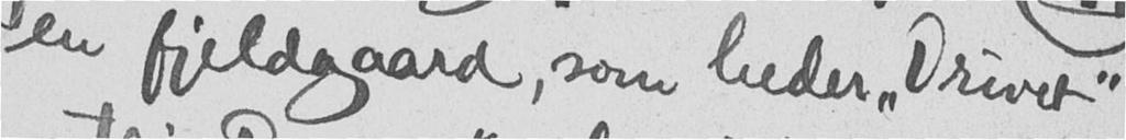en fjeldgaard, som heder "Drivet"
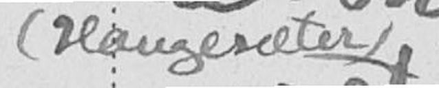(Haugesæter)
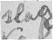slag
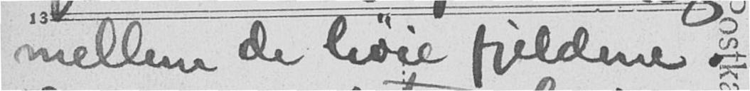mellem de høie fieldene.
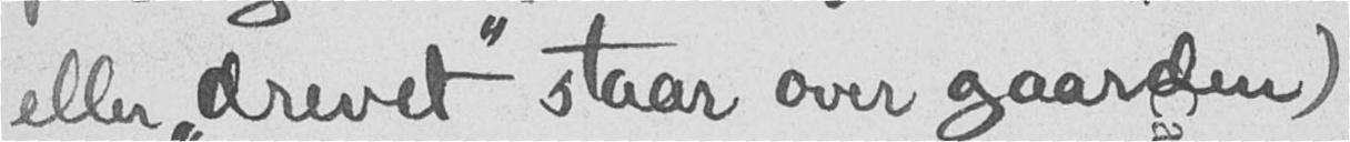eller "drevet" staar over gaarden).
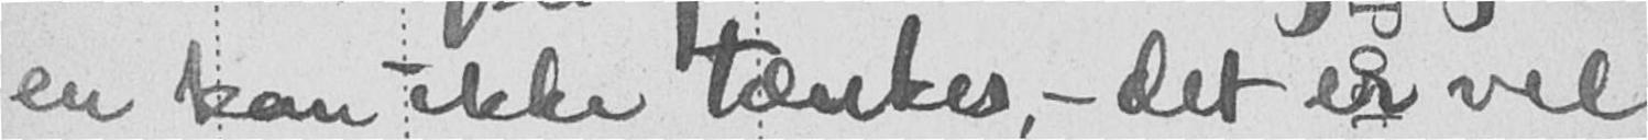en kan ikke tænkes, - det er vel
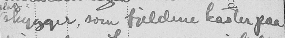skygger, som fjeldene kaster paa
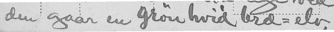den gaar en grøn hvid bræ-elv,
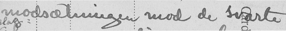modsætningen mod de svarte
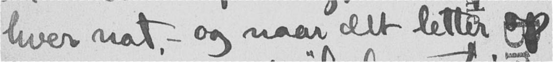hver nat - og naar det letter op
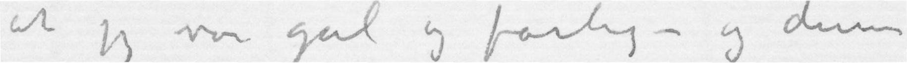at jeg var gal og farlig – og denne
